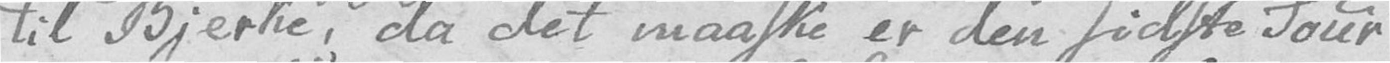til Bjerke, da det maaske er den sidste Tour
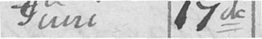Juni 17de
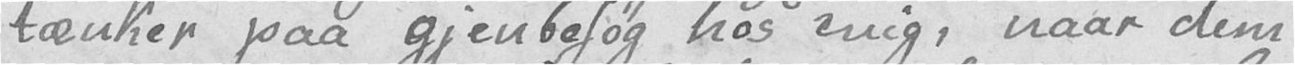tænker paa gjenbesøg hos mig, naar dem
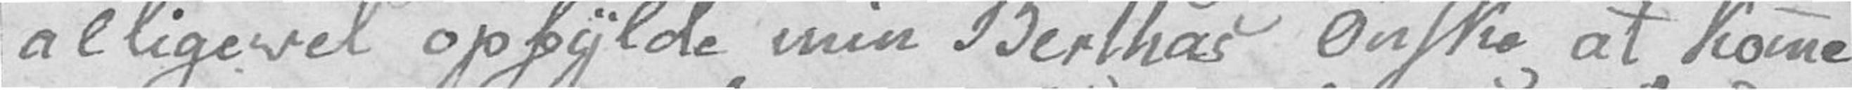alligevel opfylde min Berthas ønske at komme
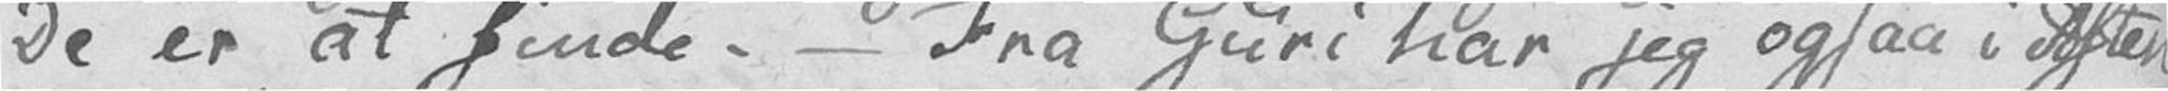De er at finde. – Fra Guri har jeg ogsaa i Aften
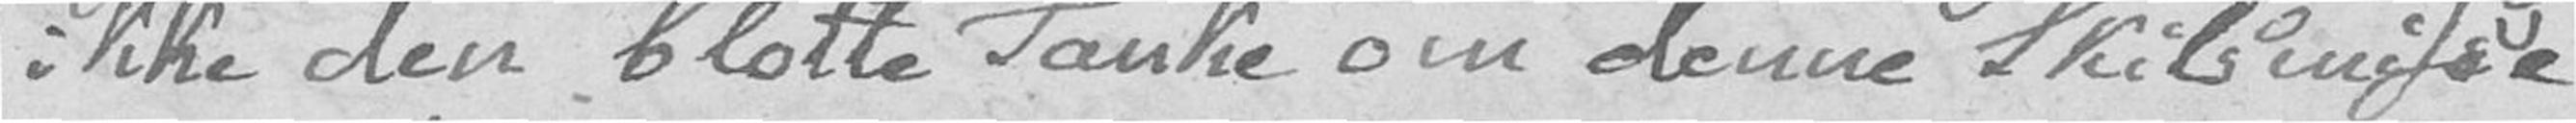ikke den blotte Tanke om denne Skilsmisse
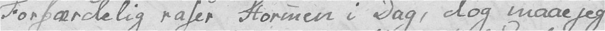Forfærdelig raser Stormen i Dag, dog maae jeg
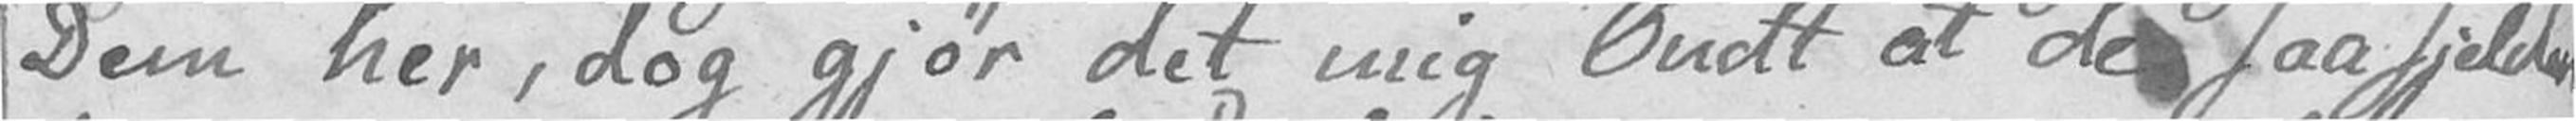Dem her, dog gjør det mig Ondt at de saa sjelden
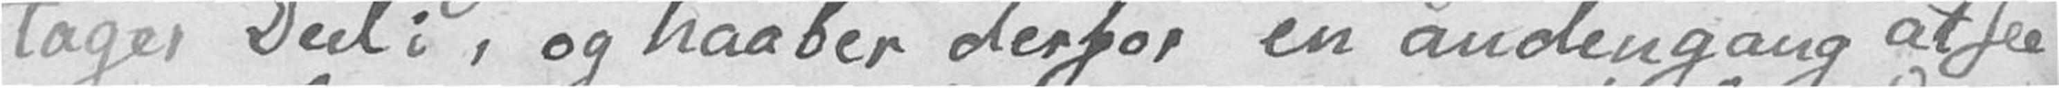tager Deel i, og haaber derfor en andengang at see
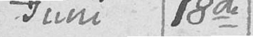Juni 18de
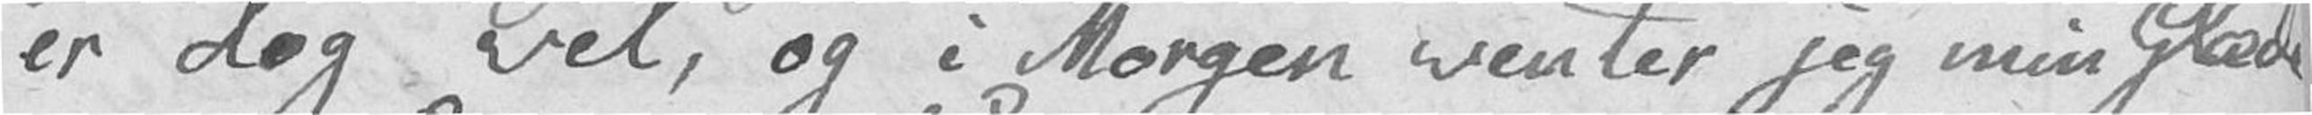er dog vel, og i Morgen venter jeg min Glæde
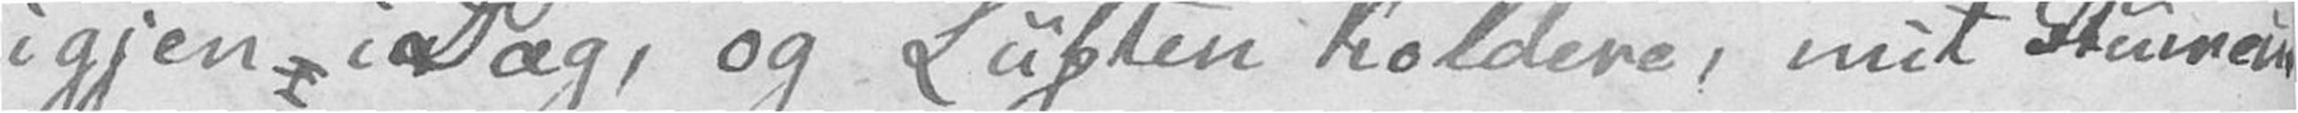igjen, i Dag, og Luften koldere, mit Humeur
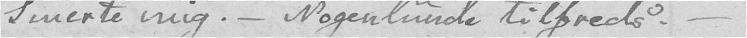Smerte mig. – Nogenlunde tilfreds. –
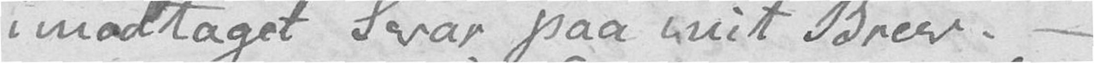imodtaget Svar paa mit Brev. –
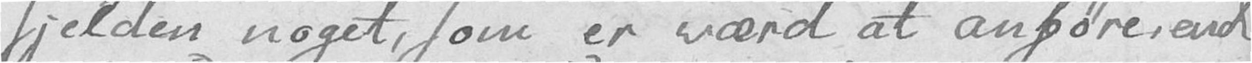sjelden noget, som er værd at anføre, end
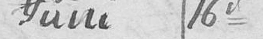Juni 16d
